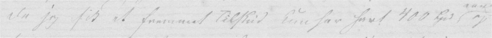da jeg fik et fremmet Tilskud kun har havt 400 Spd og
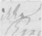ikke
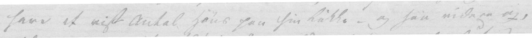have et vist Antal Høns paa sin Løkke – og saa videre op,
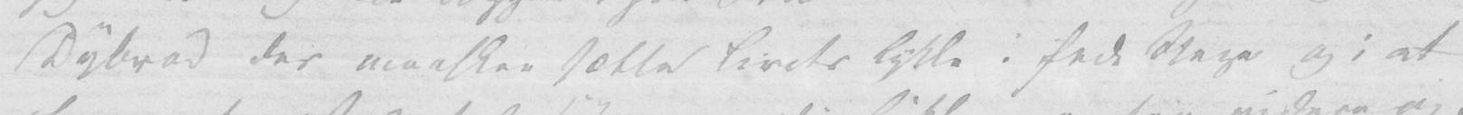Dybwad der maaskee sætte Livets Lykke i fede Stege og i at
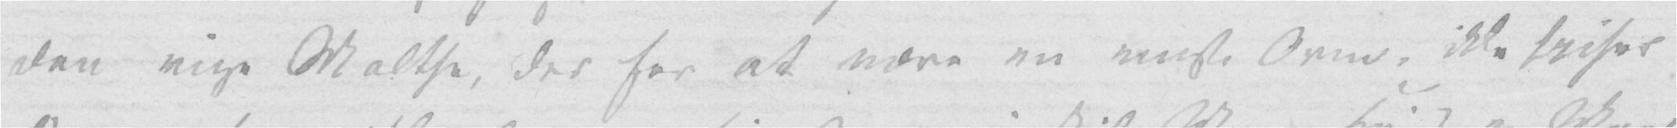den rige Malthe, der for at nære en eneste Orm, ikke spiser
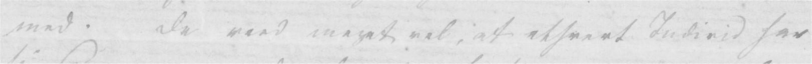med. De veed meget vel, at ethvert Individ har
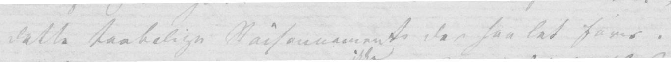dette taabelige Raisonnement der saa let føres
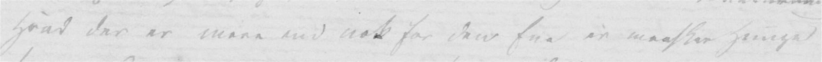Hvad der er mere end nok for den Ene er maaskee Hunger
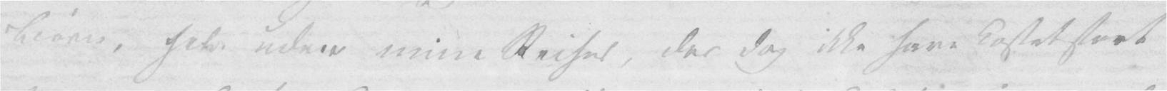Børn, selv uden mine Reiser, der dog ikke have kostet stort
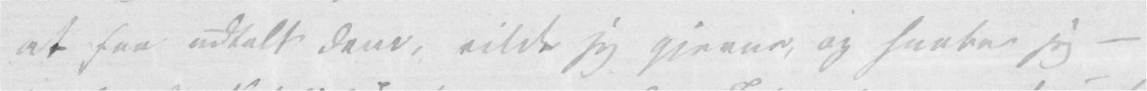at faa udtalt dem, vilde jeg gjerne, og haaber jeg –
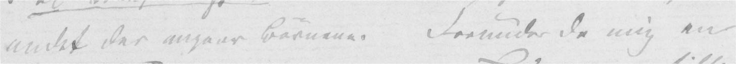andet der angaar Børnene. Forunder De mig en
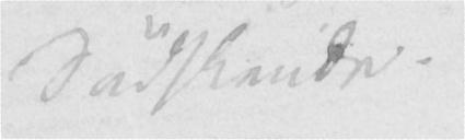Sødskende.
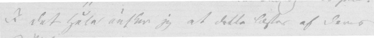I det Hele ønsker jeg at dette læstes af Deres
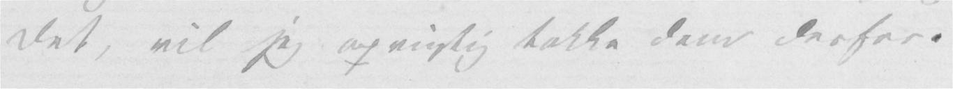det, vil jeg oprigtig takke Dem derfor.
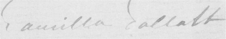Camilla Collett
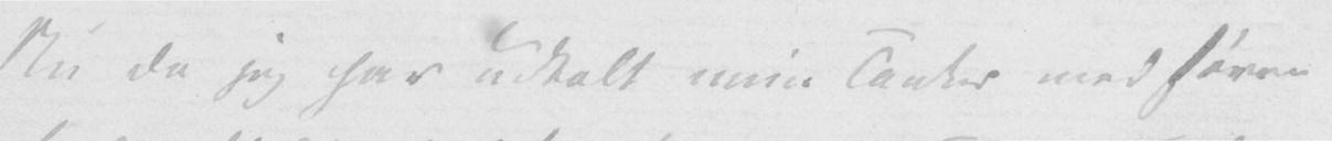Nu da jeg har udtalt mine Tanker med større
